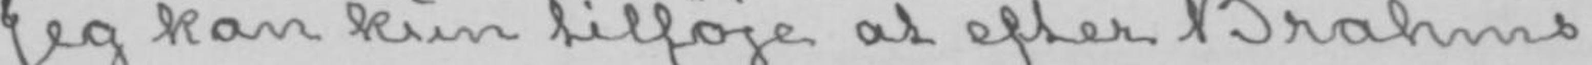Jeg kan kun tilføje at efter Brahms
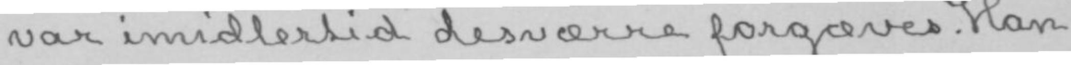var imidlertid desværre forgæves. Han
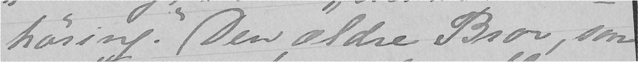høring. Den ældre Bror, som
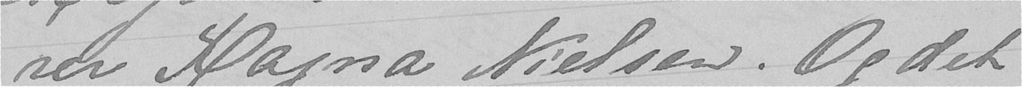rer Ragna Nielsen. Og det
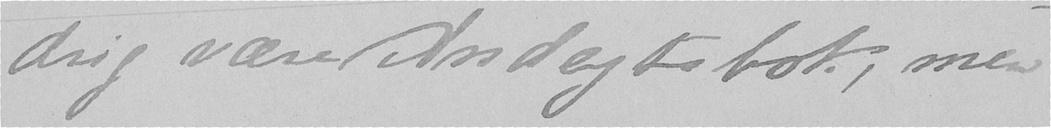drig være Andagtsbok; men
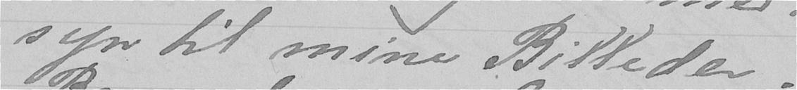syn til mine Billeder.
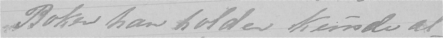Boken han holder kunde al-
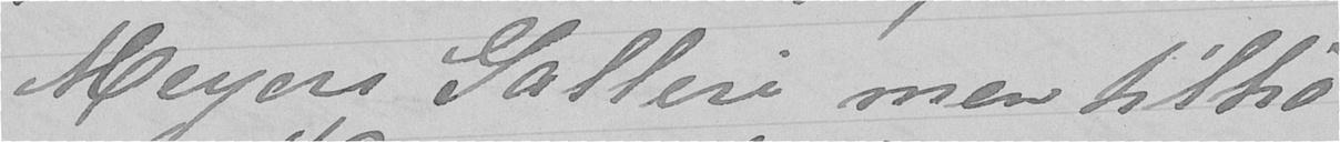Meyers Galleri men tilhø-
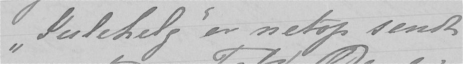Julehelg er netop sendt
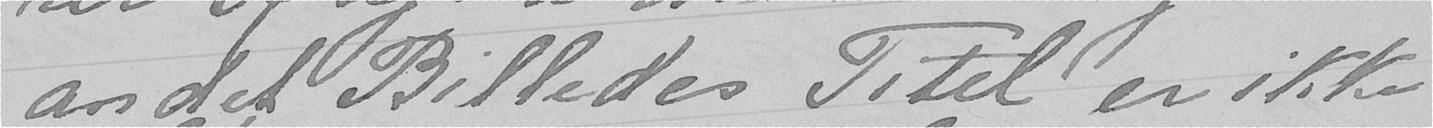andet Billedes Titel er ikke
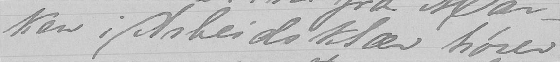ken i Arbeidsklær hører
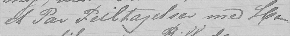et Par Feiltagelser med Hen-
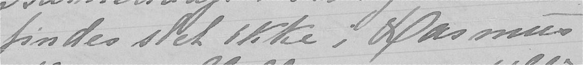findes slet ikke i Rasmus
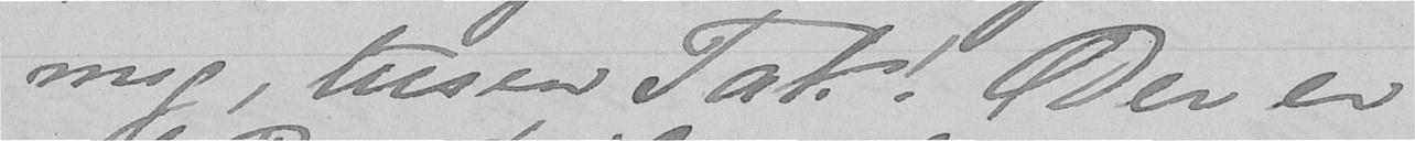mig, tusen Tak! Der er
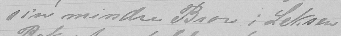sin mindre Bror i Leksene
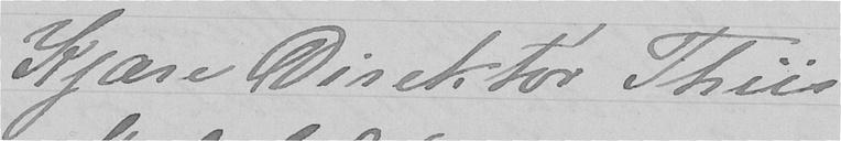Kjære Direktør Thiis
